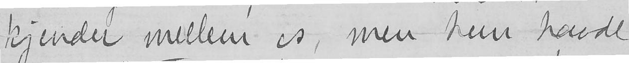kjender mellem os, men hun havde
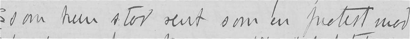som hun stog rent som en protest mod
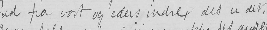ud fra vort og eders indre, det er det,
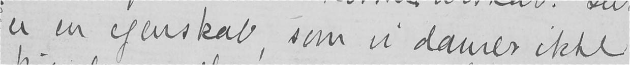er en egenskab, som vi damer ikke
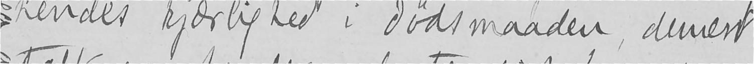hendes kjærlighed i dødsmaaden, dernest
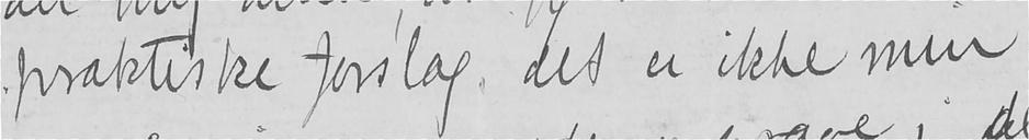praktiske forslag, det er ikke min
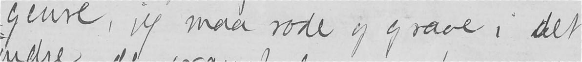genre, jeg maa rode og grave i det
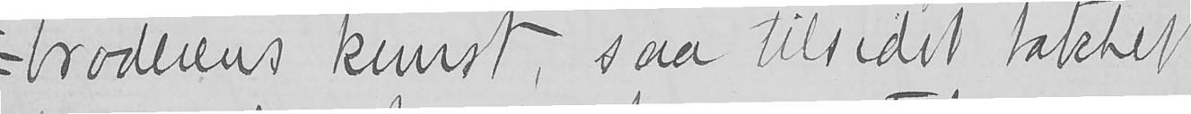broderens kunst, saa tilsidst takket
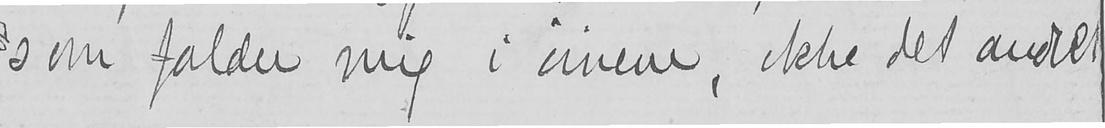som falder mig i øinene, ikke det andet
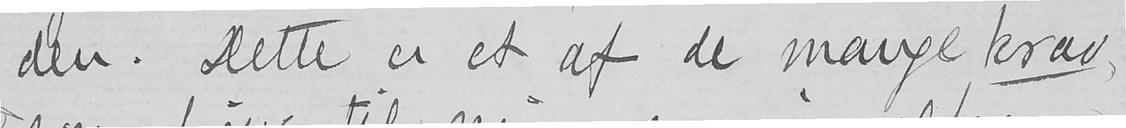den. Dette er et af de mange krav,
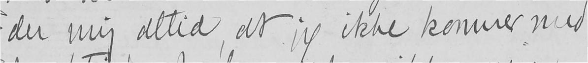der mig altid, at jeg ikke kommer med
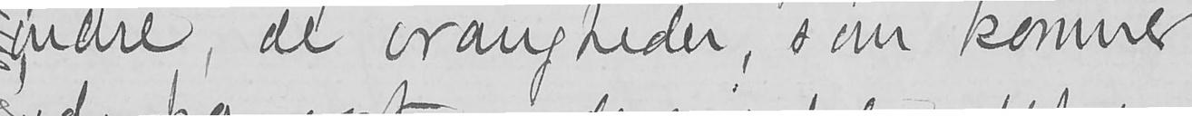indre, de vrangheder, som kommer
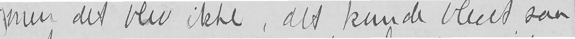men det blev ikke, det kunde blevet saa
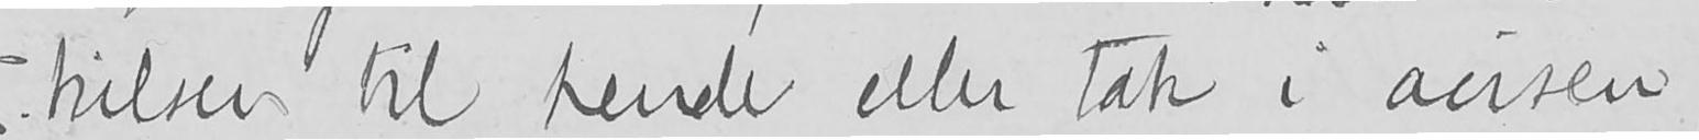hilsen til hende eller tak i avisen,
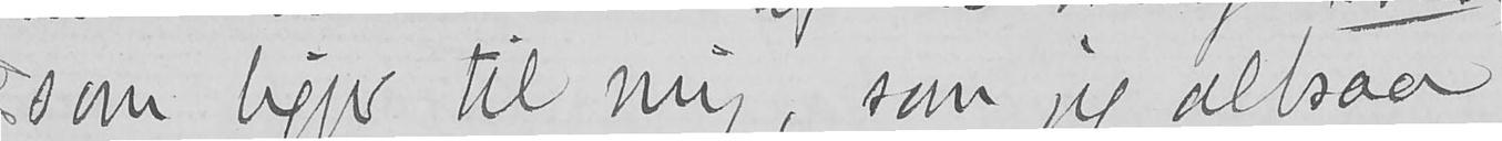som ligger til mig, som jeg altsaa
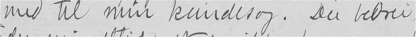med til min kvindesag. Du bebrei-
